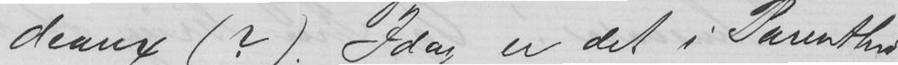deaux. Idag er det i Parenthes
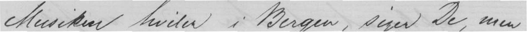Musiken hviler i Bergen, siger De, men
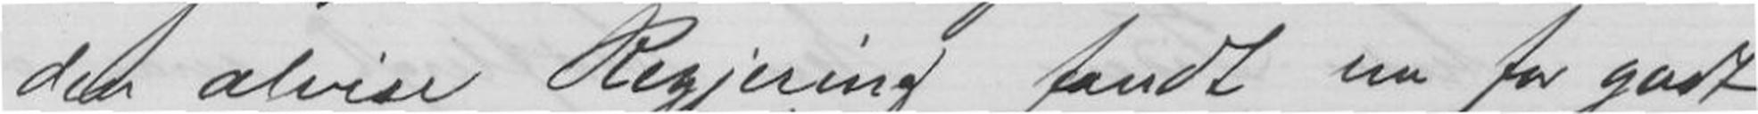den alvise Regjering fandt nu for godt
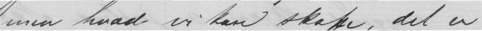men hvad vi kan skaffe, det er
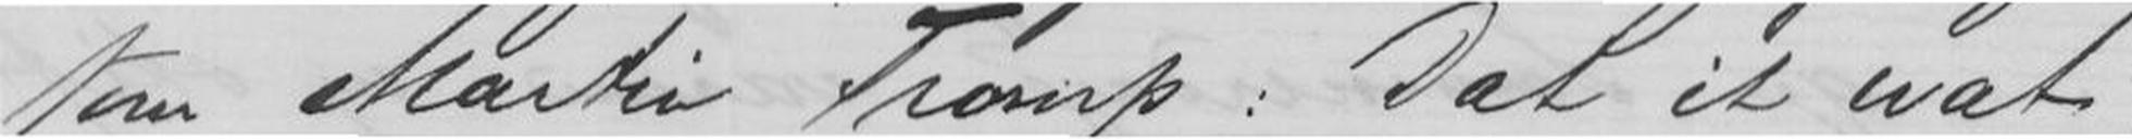som Martin Tramp: Dat it wat
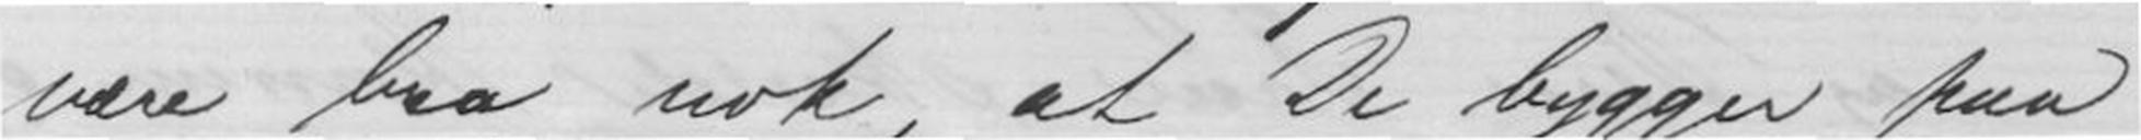være bra nok, at De bygger paa
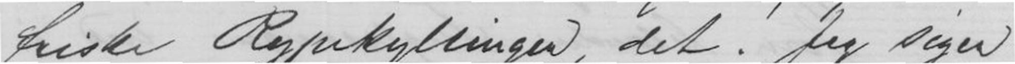freske Rypekyllinger, det! Jeg siger
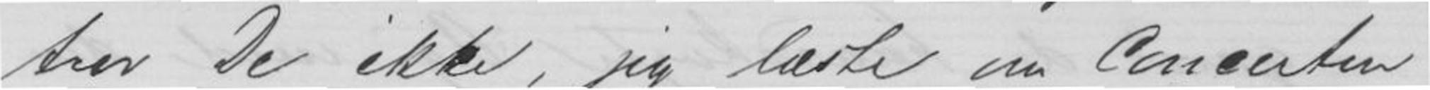tror De ikke, jeg læste om Concerten
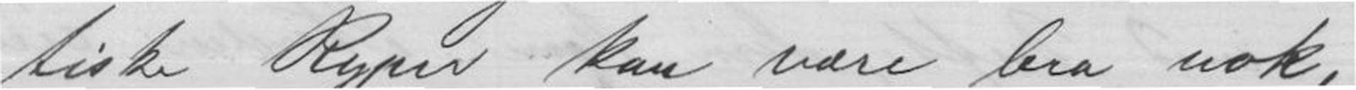tiske Ryper kan være bra nok, -
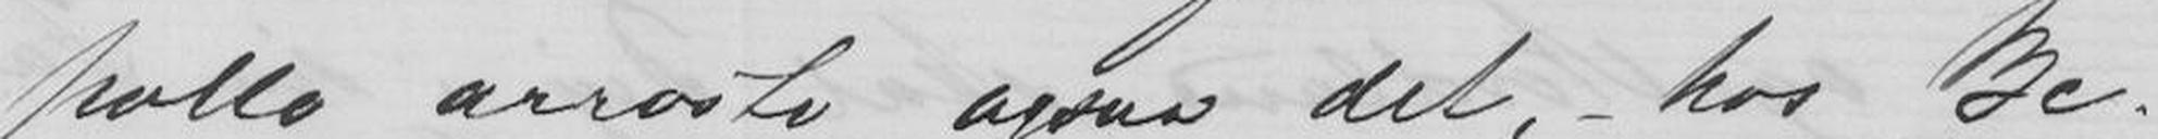pollo arrosto ogsaa det, - hos Be-
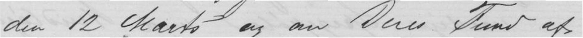den 12 Marts og om Deres Fund af
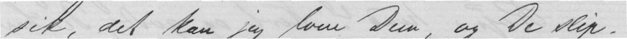sik, det kan jeg love Dem, og De slip-
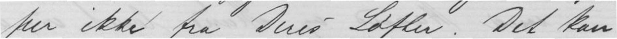per ikke fra Deres Løfter. Det kan
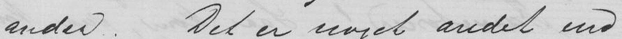ander. Det er noget andet end
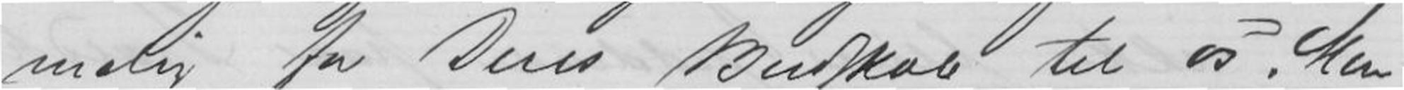melig for Deres Budskab til os. Men
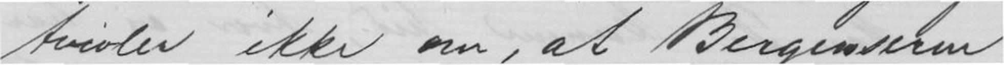tvivler ikke om, at Bergenseren
